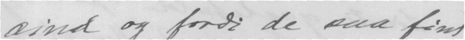sind og fordi de saa fint
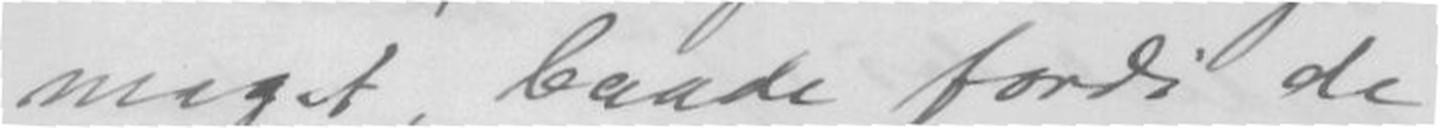meget, baade fordi de
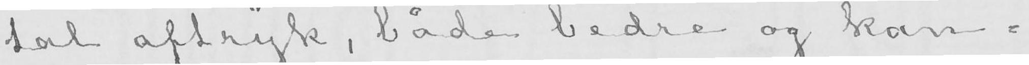tal aftryk, både bedre og kan-
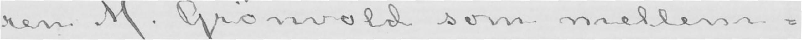ren M. Grønvold som mellem-
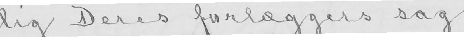lig Deres forlæggers sag.
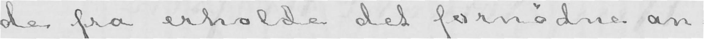de fra erholde det fornødne an-
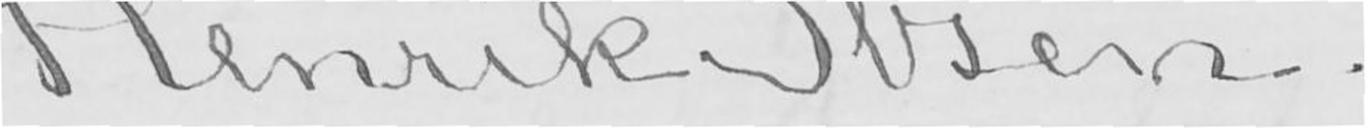Henrik Ibsen.
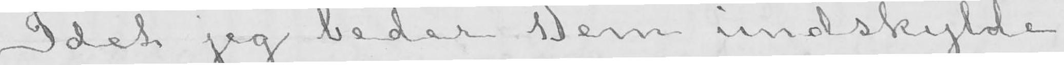Idet jeg beder Dem undskylde
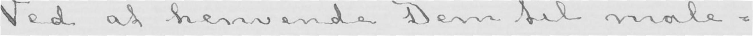Ved at henvende Dem til male-
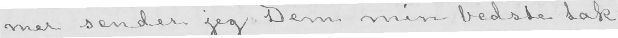mer sender jeg Dem min bedste tak
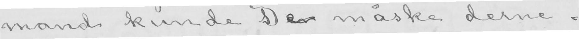mand kunde De måske derne-
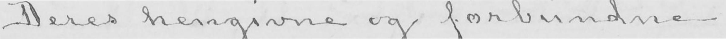Deres hengivne og forbundne
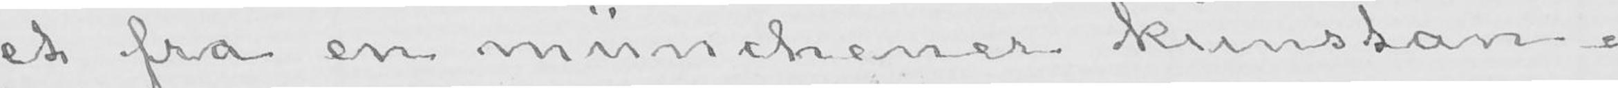et fra en münchener kunstan-
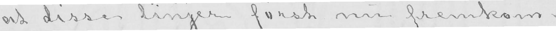at disse linjer først nu fremkom-
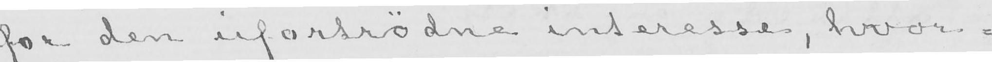for den ufortrødne interesse, hvor-
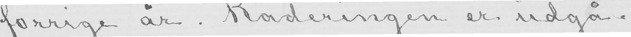forrige år. Raderingen er udgå-
